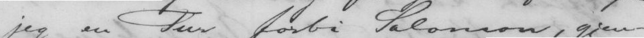jeg en Tur forbi Salomon, gjen-
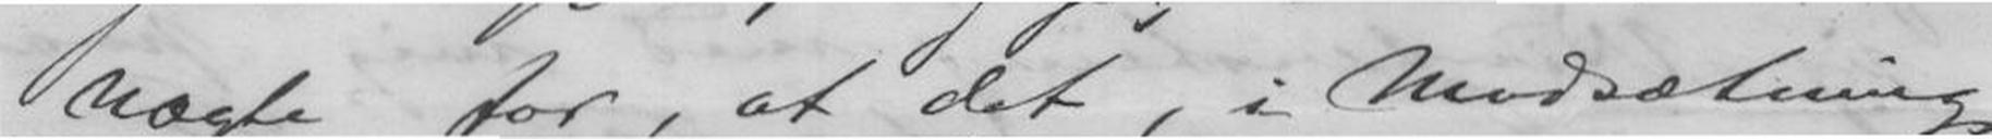nægte for, at det, i Modsætning
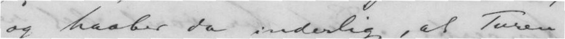og haaber da inderlig, at Turen
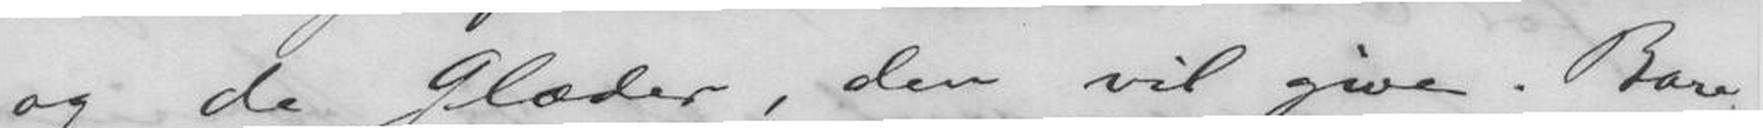og de Glæder, den vil give. Bare
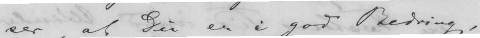Jeg ser, at Du er i god Bedring,
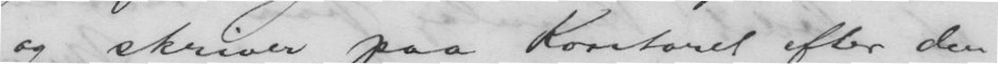og skriver paa Kontoret efter den
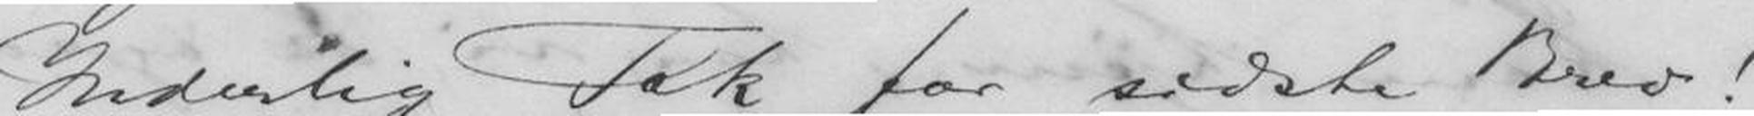Inderlig Tak for sidste Brev!
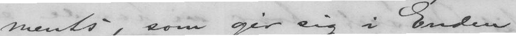ments, som gir sig i Enden,
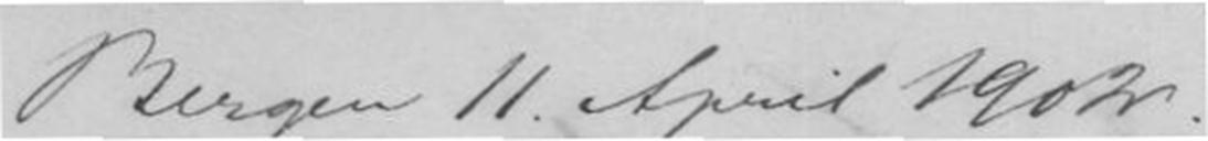Bergen 11. April 1902.
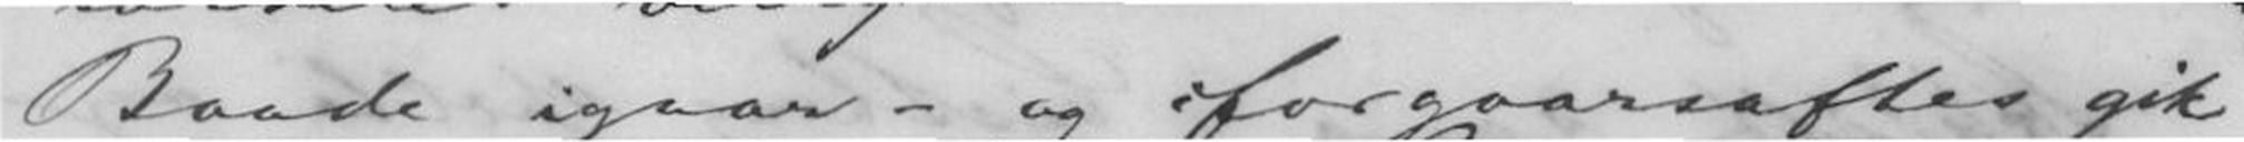Baade igaar- og iforgaarsaftes gik
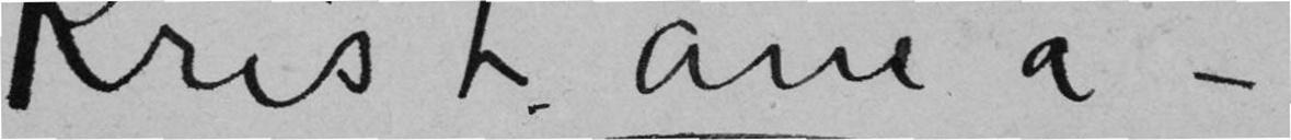Kristiania –
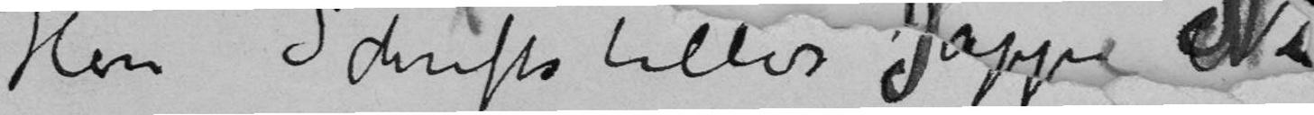Hrn Schriftsteller Jappe N
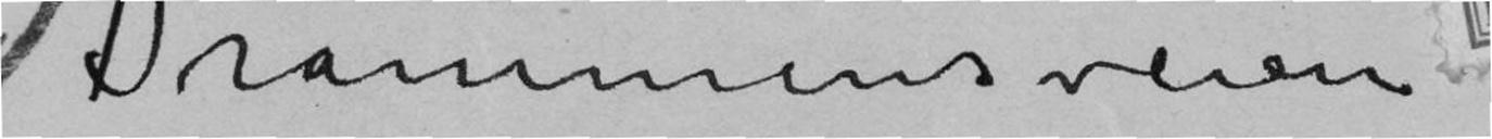Drammensveien
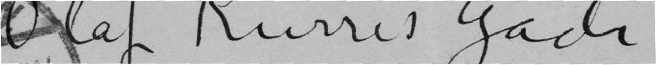Olaf Kürres gade
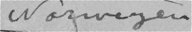Norwegen
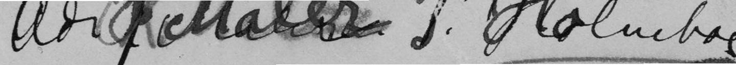AdrMaler T. Holmboe
